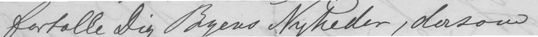fortælle Dig Byens Nyheder, dersom
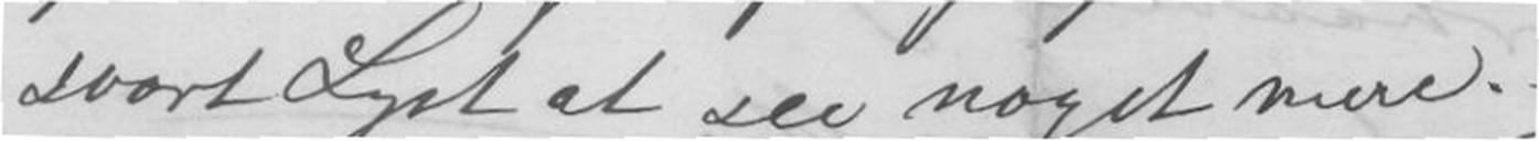svært Lyst at see noget mere.
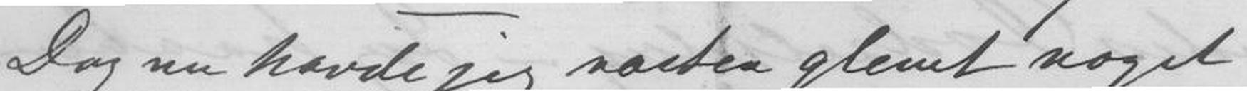Dog nu havde jeg næsten glemt noget
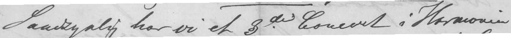Sandsynlig har vi et 3die Concert i Harmonien
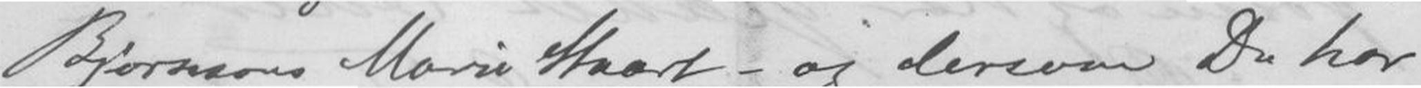Bjørnsons Marie Stuart - og dersom Du har
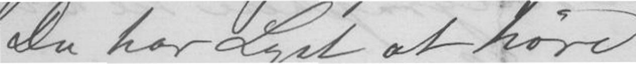Du har Lyst at høre
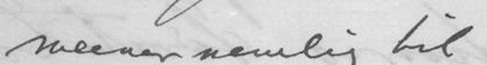meener nemlig til
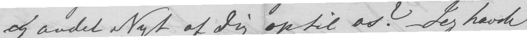og andet Nyt af Dig optil os? - Jeg havde
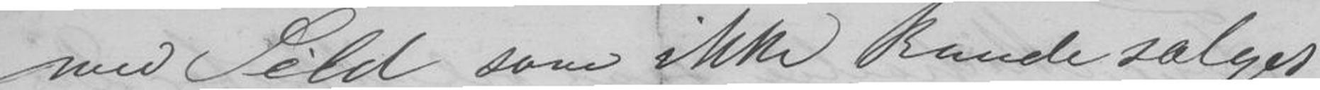med Sild som ikke kunde sælges
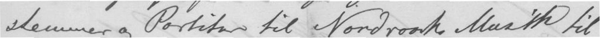stemmer og Partitur til Nordraak Musik til
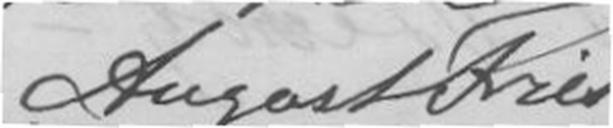August Fries.
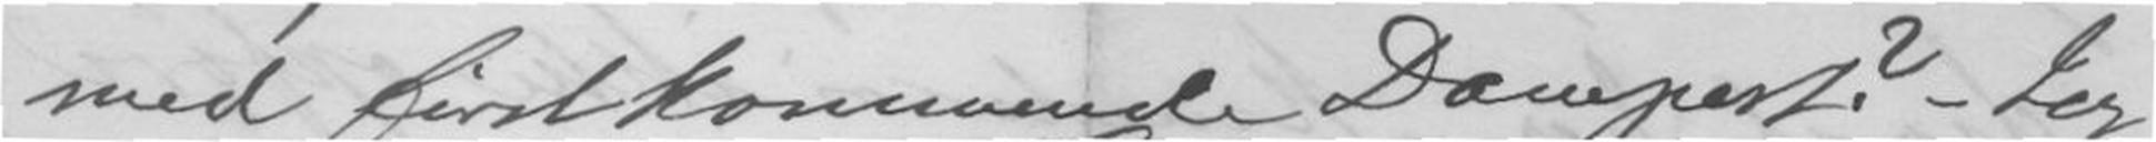med førstkommende Dampost? - Jeg
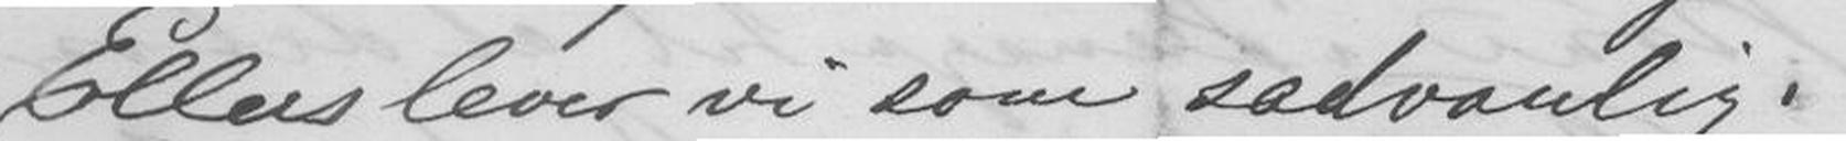Ellers lever vi som sædvanlig.
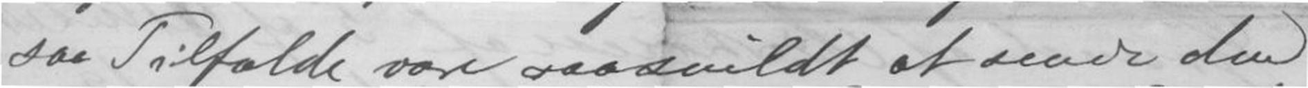saa Tilfælde være saasnildt at sende den
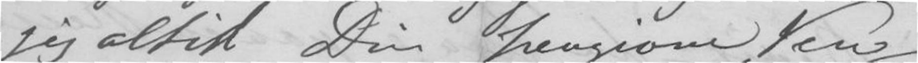jeg altid Din hengivne Ven.
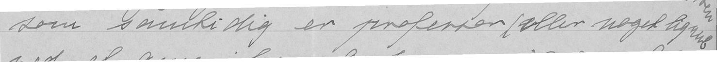som samtidig er professor (eller noget lignende
